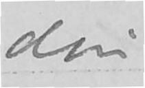din
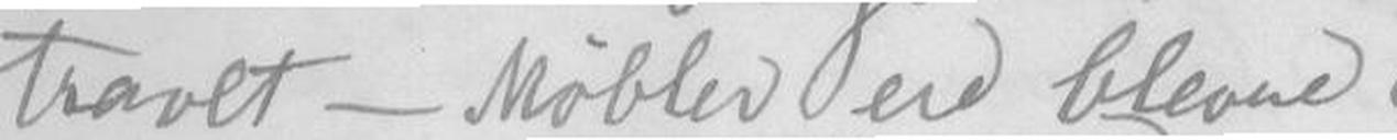travelt - Møbler ere blevne
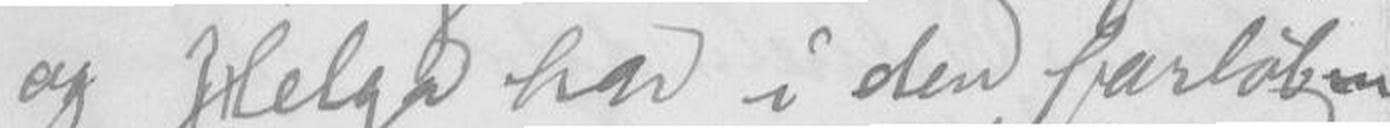og Helga har i den forløbne
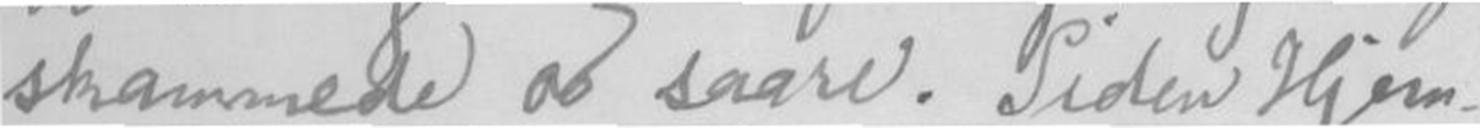skammede os saare. Siden Hjem-
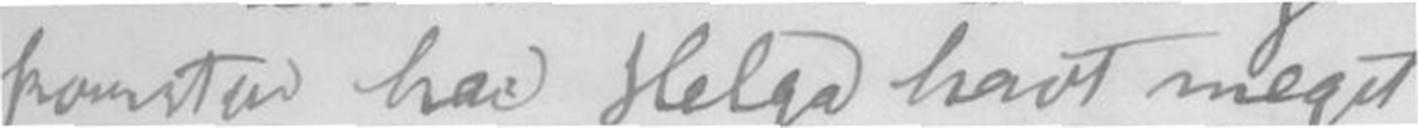komsten har Helga havt meget
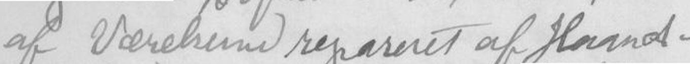af Værelsene repareret af Haand-
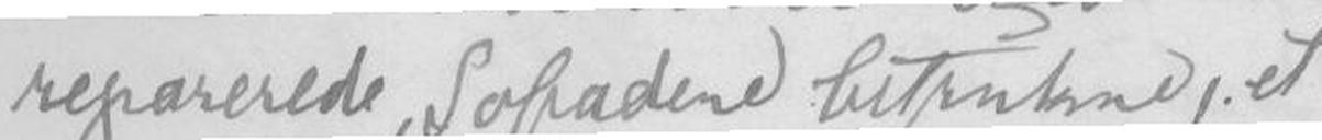reparerede, Sofadene betrukne, et
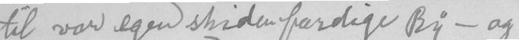til vor egen skidenfærdige By - og
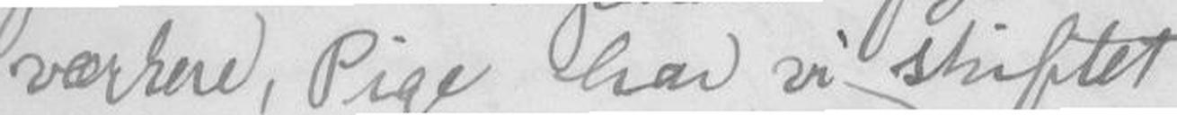værkere, Pige har vi skiftet,
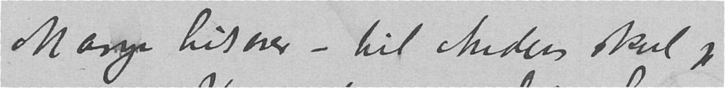Mange hilsener – til Anders skal jeg
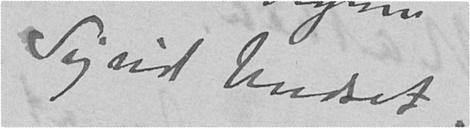Sigrid Undset.
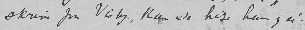skrive fra Visby, kan De hilse ham og si.
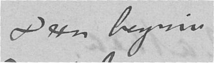Deres hengivne
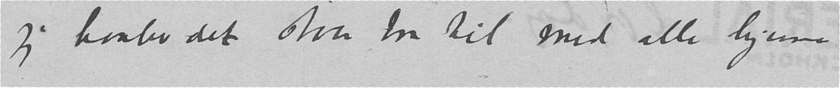Jeg haaber det staar bra til med alle hjemme
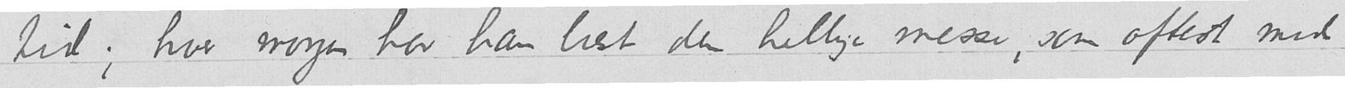tid; hver morgen har han læst den hellige messe, som oftest med
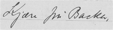Kjære fru Backer,
